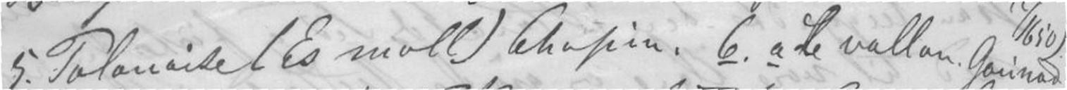5. Polonaise Es moll) Chopin. 6 a Le vollan Gounod.
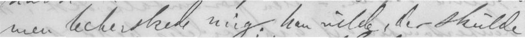men beherskede mig; han vilde, der skulde
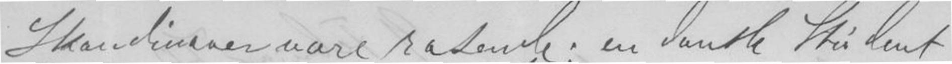Skandinaver vare rasende; en dansk Student
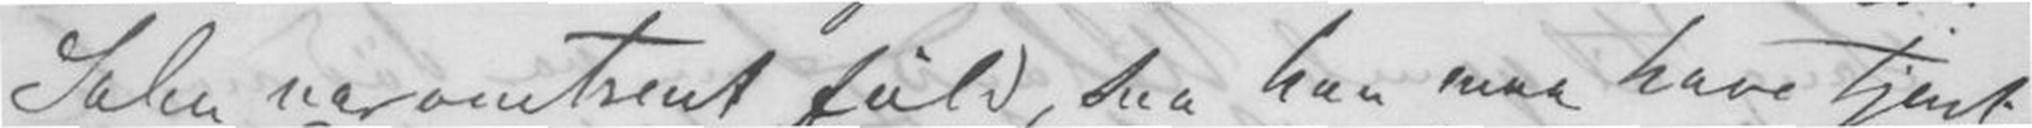Salen var omtrent fuld, saa han maa have tjent
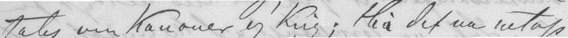tales om Kanoner og Krig; thi det var netop
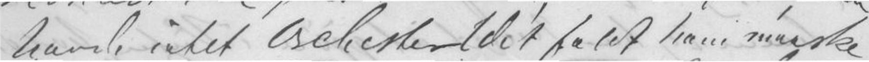havde intet Orchester (det falt ham maaske
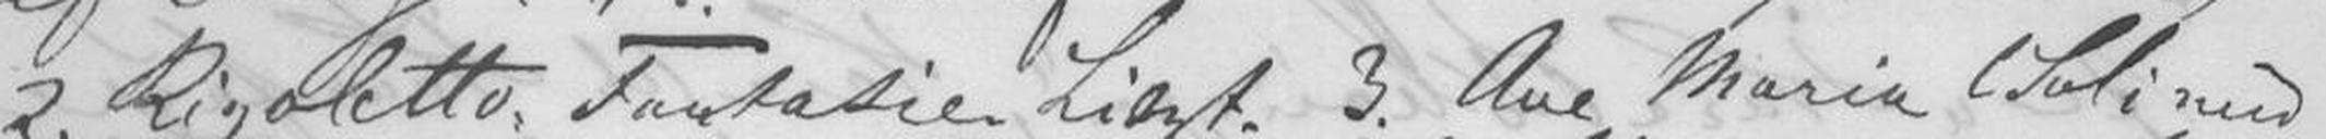2. Rigoletto, Fantasie Liszt. 3. Ave Maria (Soli med
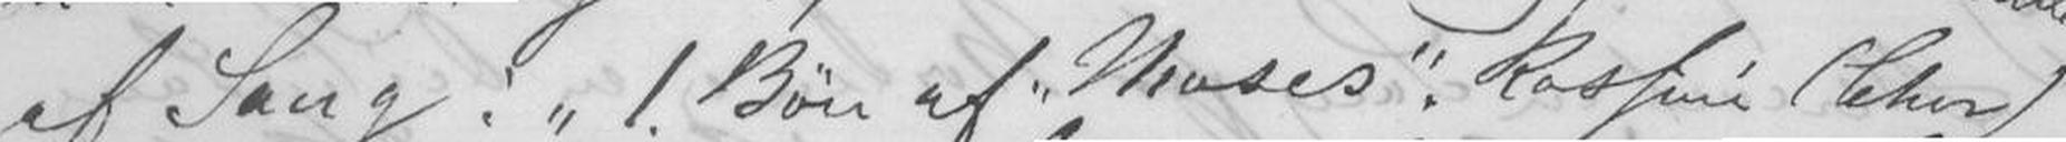af Sang: "1. Bøn af Moses. Rossini (Chor)
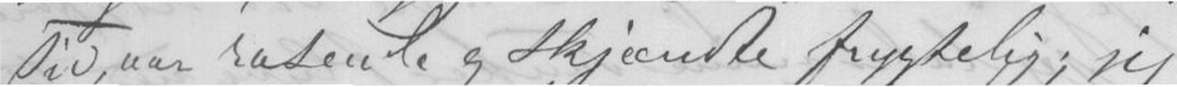Tid, var rasende og skjændte frygtelig; jeg
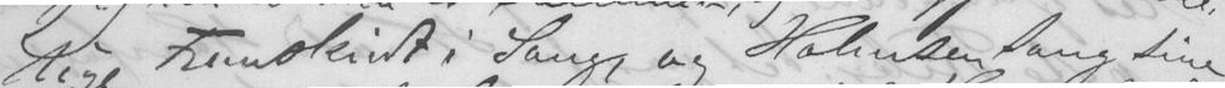lige Fremskridt i Sang og Holmsen sang sine
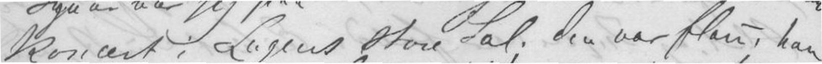koncert i Logens store Sal; den var flau; han
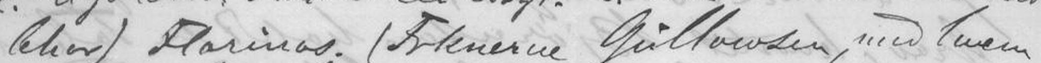Chor) Florinos. (Frknerne Gullowsen, med hvem
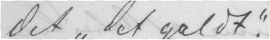detdet galdt.
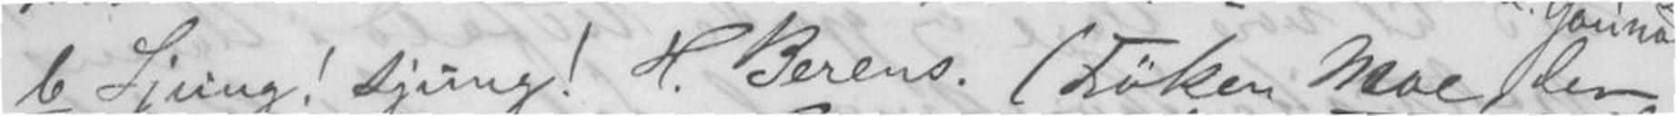b Sjung! sjung! H. Berens. (Frøken Moe, der
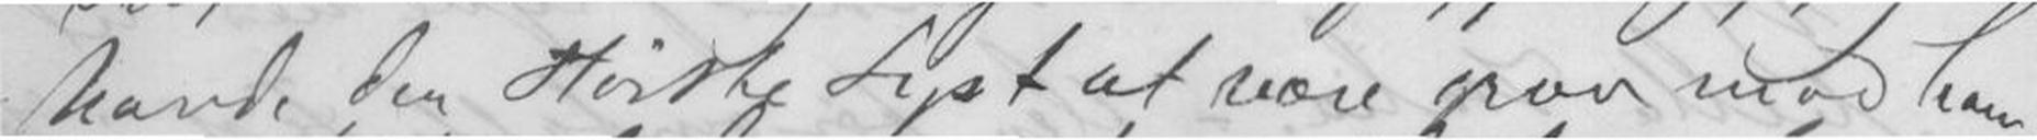havde den største Lyst at være grov mod ham,
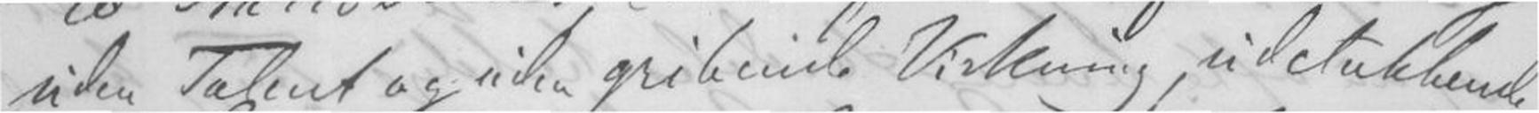uden Talent og uden gribende Virkning, udelukkende
